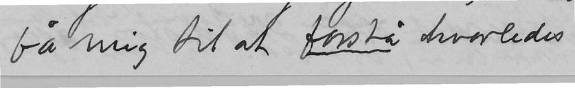få mig til at forstå hvorledes
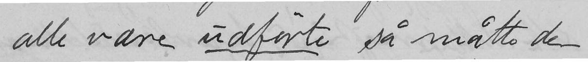alle være udførte så måtte der
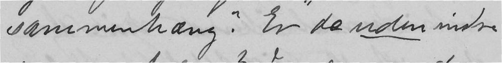sammenhæng". Er de uden indre
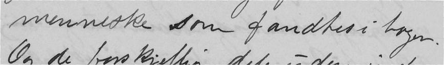menneske som fandtes i bogen.
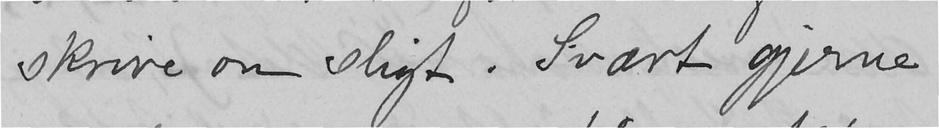skrive om sligt. Svært gjerne
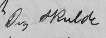Du skulde
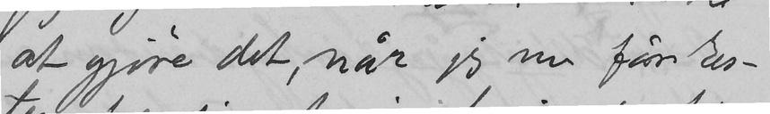at gjøre det, når jeg nu får res-
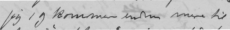jeg, og kommer endnu mere til
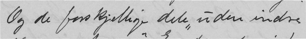Og de forskjellige dele "uden indre
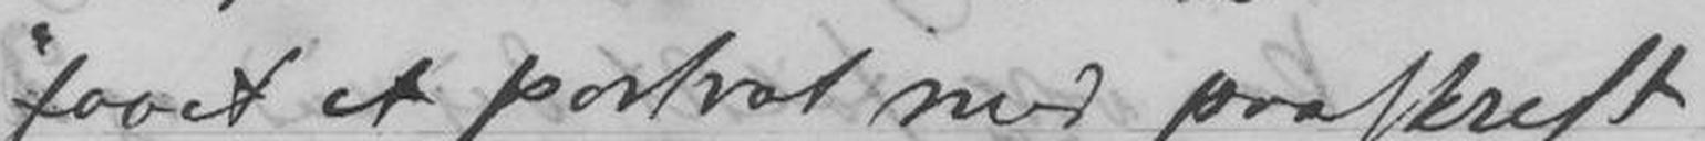faaet et portræt med paaskrift
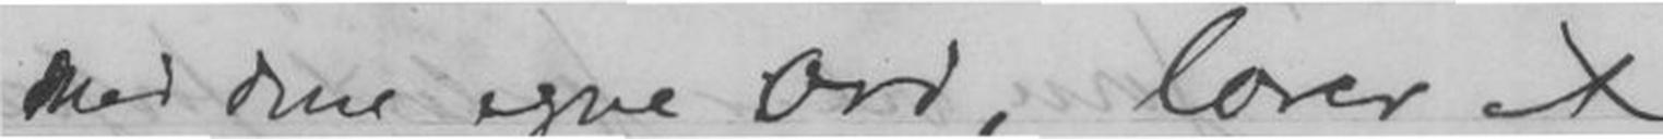med dine egne Ord, lover at
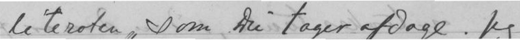leteroten, som Du tager afdage. Jeg
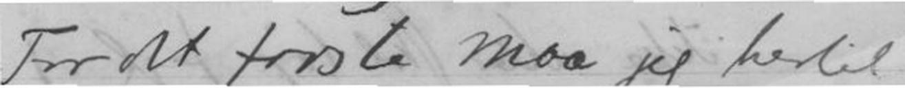For det første maa jeg hertil
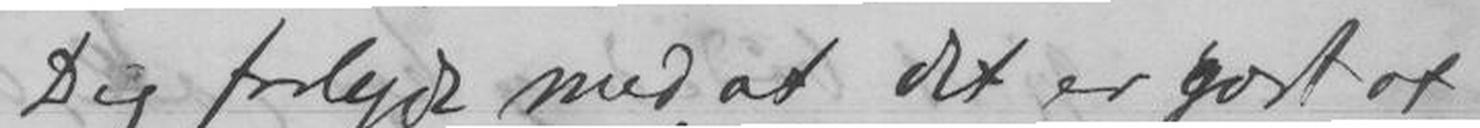Dig forlyde med, at det er godt at
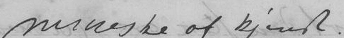menneske at kjende.
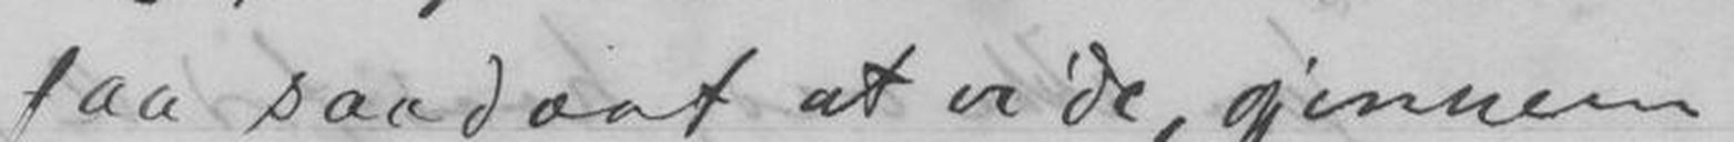faa saadant at vide, gjennem
